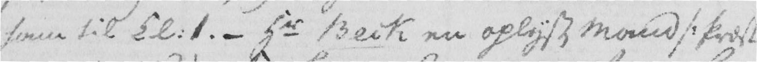ham til Kl. 1. - Hr Beck en oplyst Mand /: Præst
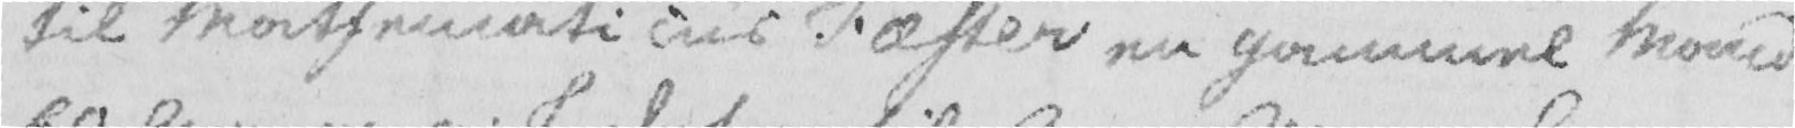til Mathematicus Fæster en gammel Mand
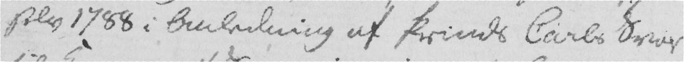selv 1788 i Anledning af Prinds Carls Svar
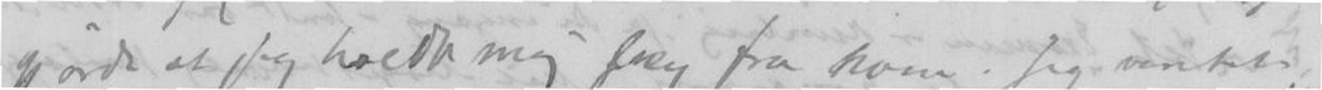gjorde at jeg holdt mig frey fra ham. Jeg ventet
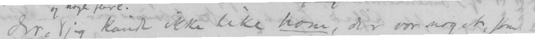der jeg kunde ikke like ham, der var noget som
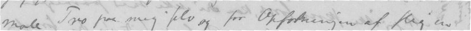male Tro paa min selv og for Opfatningen af slig en
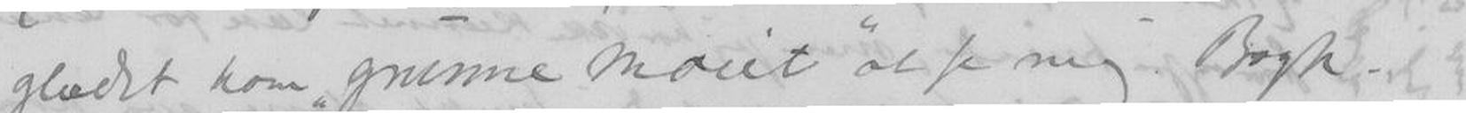glædet kom grunne Marit at se mig. Bogtr.
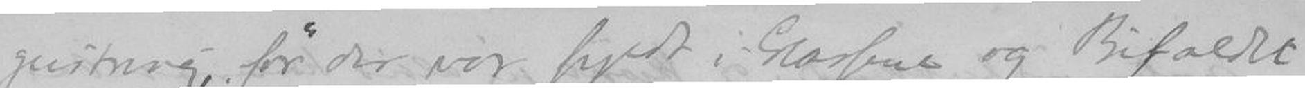geistring, før der var fyldt i Glasserne og Bifaldet
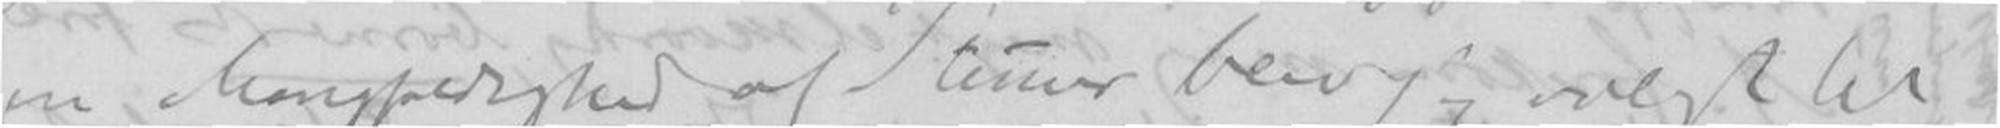en Mangfoldighed af Stemmer blev jeg valgt til
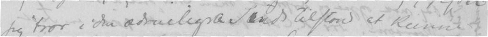jeg tror i den ædrueligste Sandtilstrand at kunne
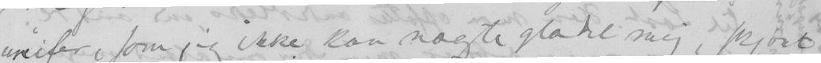umfer, som jeg ikke kan nægte glædet mig, skjønt
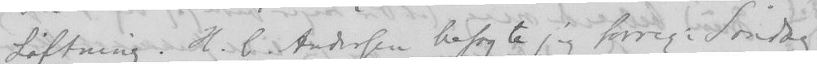Løftning. H.C.Andersen besøgte jeg forrige Søndag
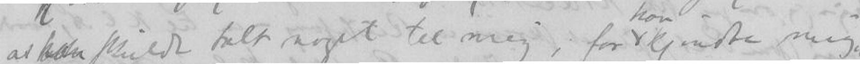at han skulde talt noget til mig, for kjendte mig
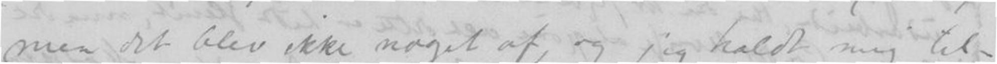men det blev ikke noget af, og jeg holdt mig til-
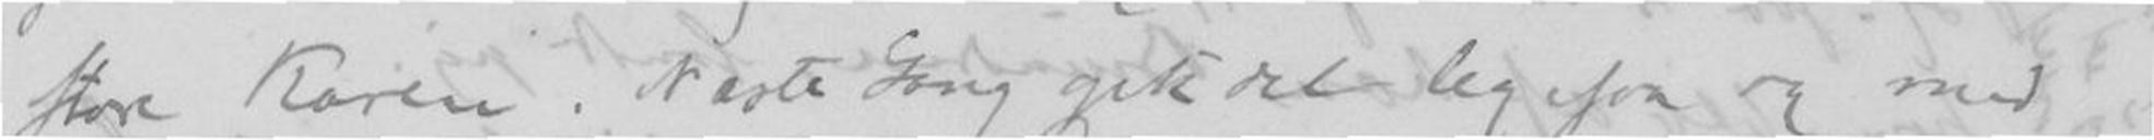store Koren. Næste Gang gik det ligesaa og med
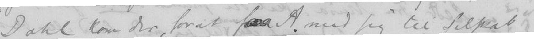Dahl kom der forat faa A. med sig til Selskab
